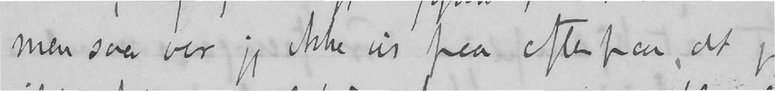men saa var jeg ikke vis paa efterpaa, at jeg
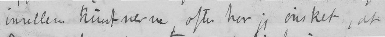imellem kunstnerne, ofte har jeg ønsket, at
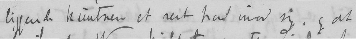liggende kunstnere et rent had imod sig, og at
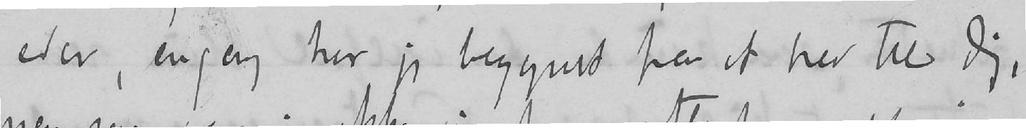eder, engang har jeg begyndt paa et brev til Dig,
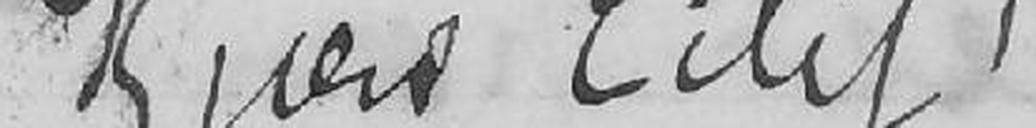Kjære Eilif!
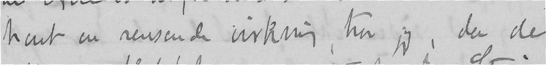havt en rensende virkning, tror jeg, da de
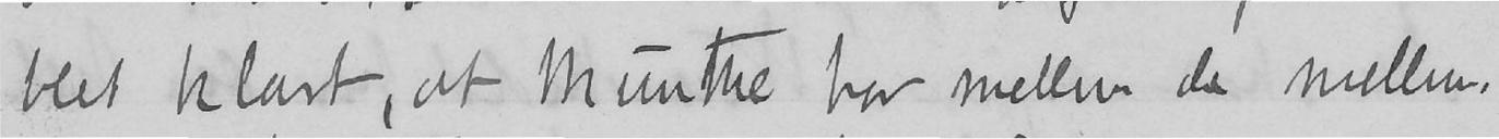blit klart, at Munthe har mellem de mellem-
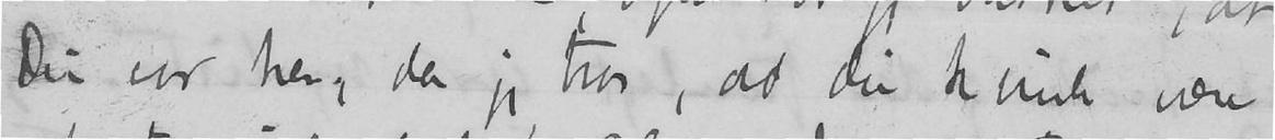Du var her, da jeg tror, at Du kunde være
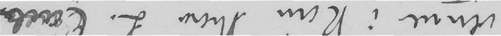venner i Rom Thorne L. Carl
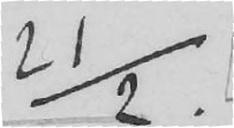21/2.
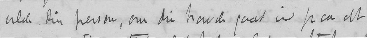vilde Din person, om du havde gaat ind paa at
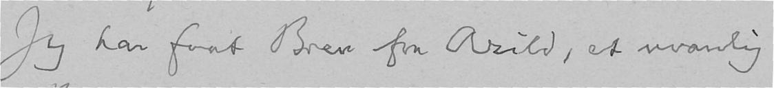Jeg har faat Brev fra Arild, et uvanlig
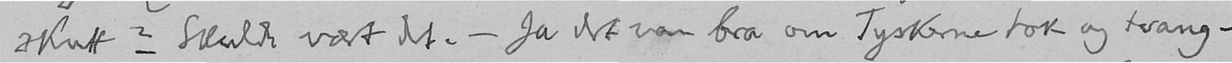skutt? Skulde vært det. - Ja det var bra om Tyskerne tok og tvang-
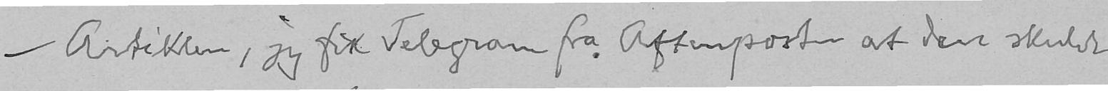- Artiklen, jeg fik Telegram fra Aftenposten at den skulde
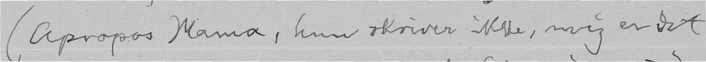(Apropos Mama, hun skriver ikke, mig er det
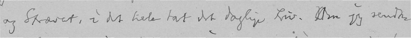og Strævet, i det hele tat det daglige Liv. Om jeg sendte
